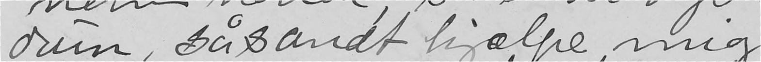dum, såsandt hælpe mig
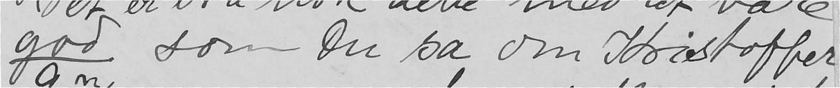god som Du sa om Kristoffer
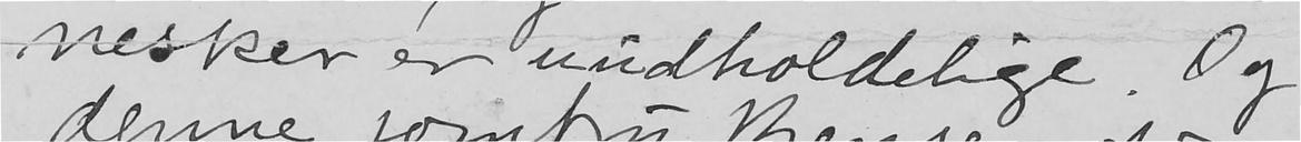nesker er uudholdelige. Og
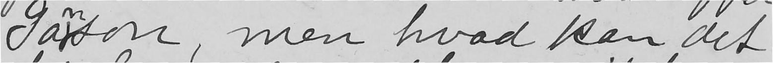Janson, men hvad kan det
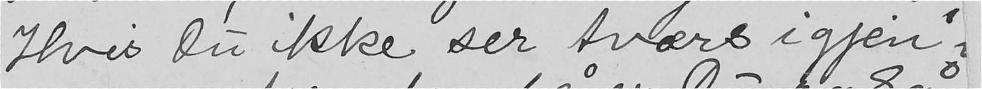Hvis Du ikke ser tværs igjen-
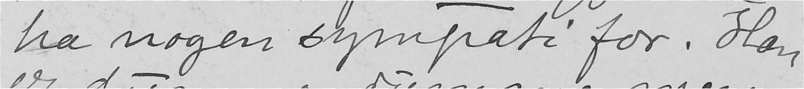ha nogen sympari for. Han
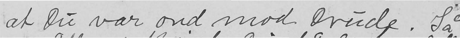at Du var ond mod Drude. Så
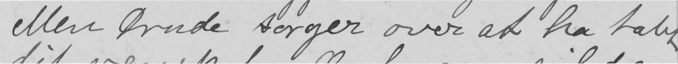Men Drude sorger over at ha tabt
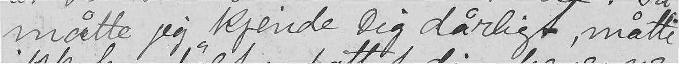måtte jeg kjende Dig dårligt, måtte
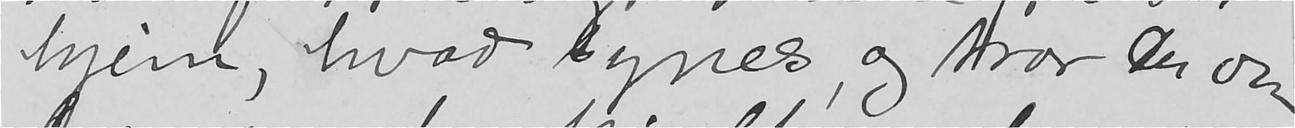hjem, hvad synes, og tror Du om
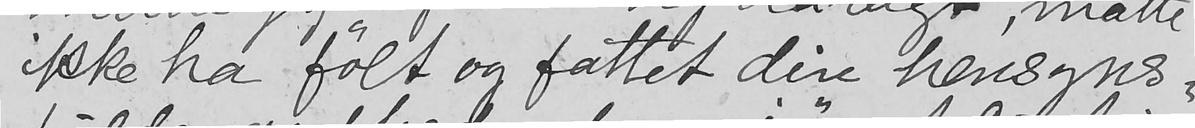ikke ha følt og fattet din hensyn-
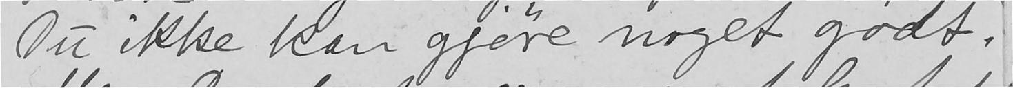Du ikke kan gjøre noget godt.
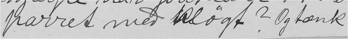parret med kløgt? Og tænk
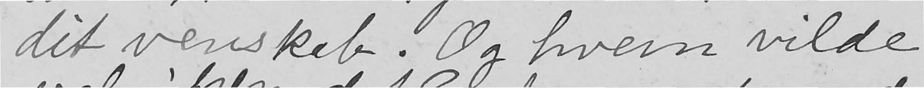dit venskeb. Og hvem vilde
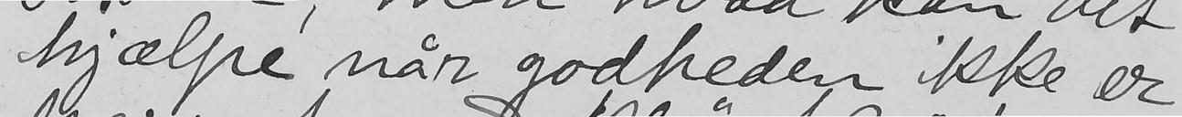hjælpe når godheden ikke er
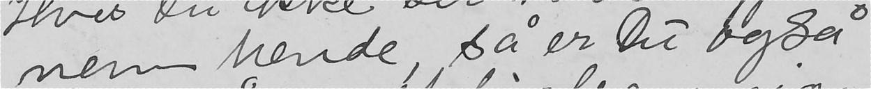nem hende, så er Du også
